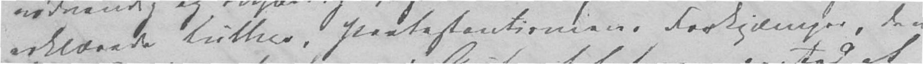erklærede Luther, Protestantismens Forkjæmper, den
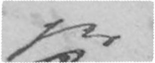ges
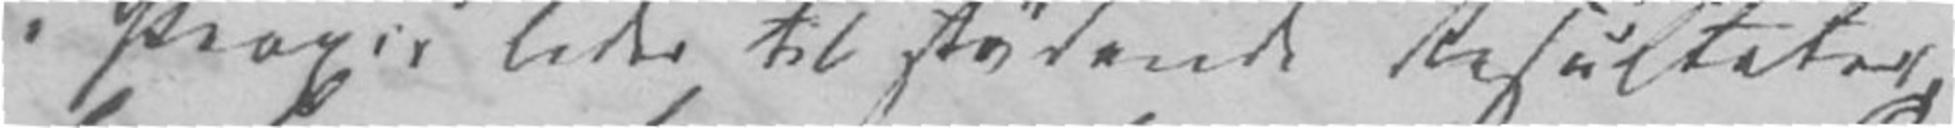i Praxis leder til stødende Resultater,
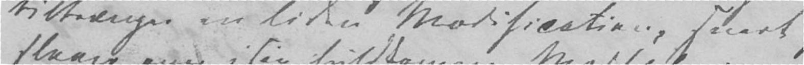tiltrænger en liden Modification, snart
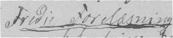Tredie Forelæsning
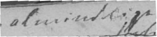almindelige
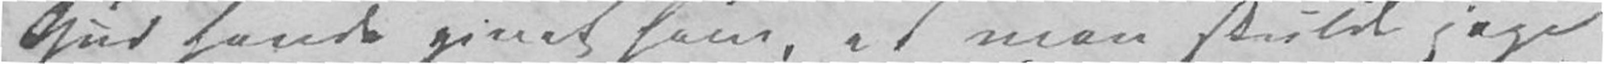Gud havde givet ham, at man skulde jage
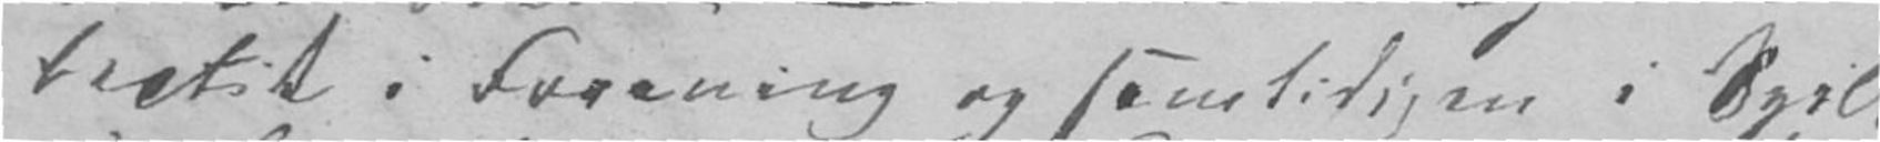lectik i Forening og samtidigen i Spil
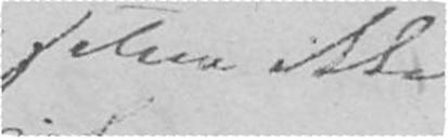selv ikke
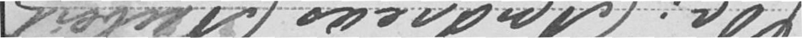Dr. Andreas Aubert
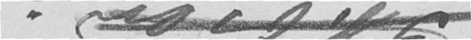Skøien.
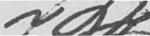Hr
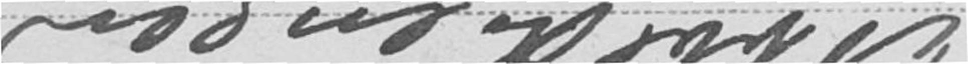Abbediengen
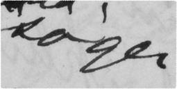søger
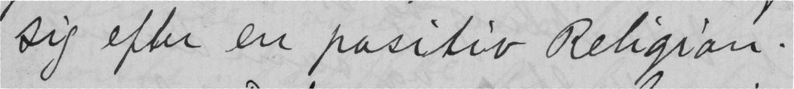sig efter en positiv Religion.
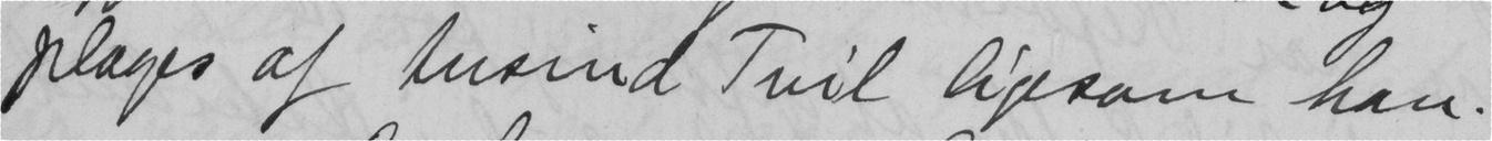plages af tusind Tvil ligesom han.
